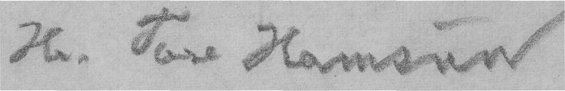Hr. Tore Hamsun
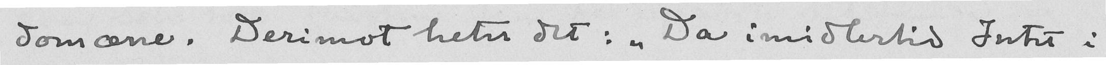domæne. Derimot heter det: "Da imidlertid Intet i
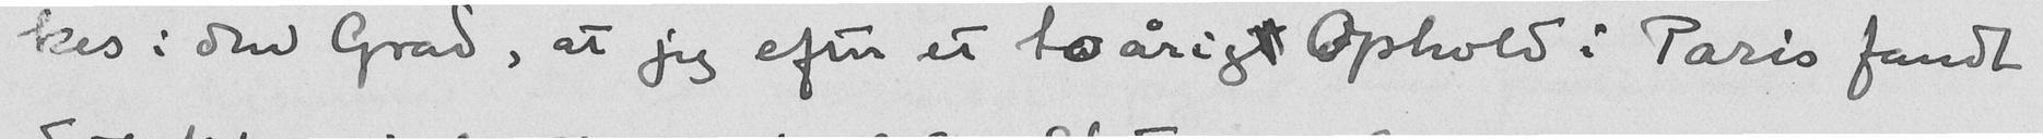kes i den Grad, at jeg efter et toårigt Ophold i Paris fandt
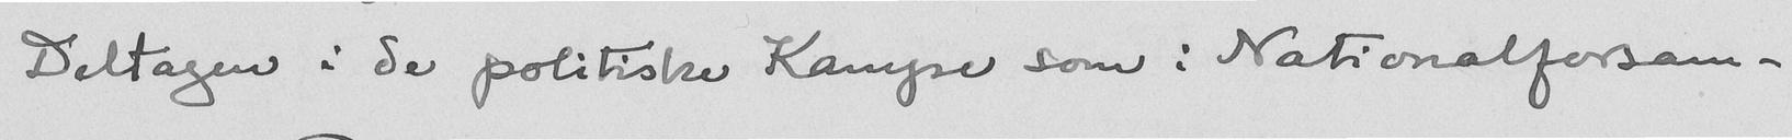Deltagen i de politiske Kampe som i Nationalforsam-
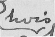hvis
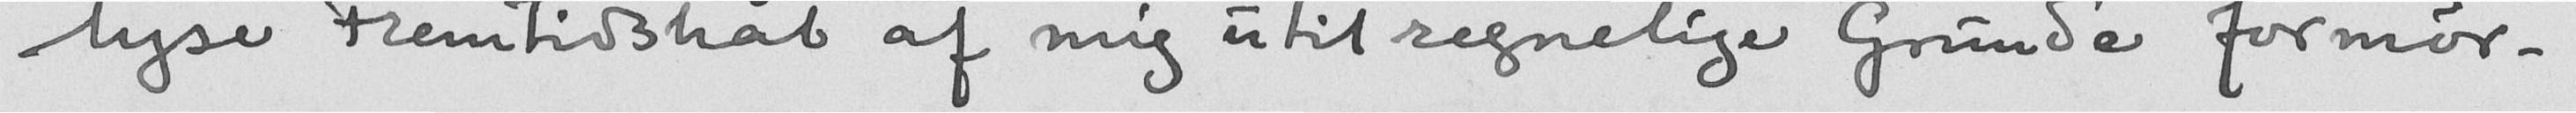lyse Fremtidshåb af mig utilregnelige Grunde formør-
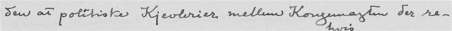den at politiske Kjevlerier mellem Kongemagten der re-
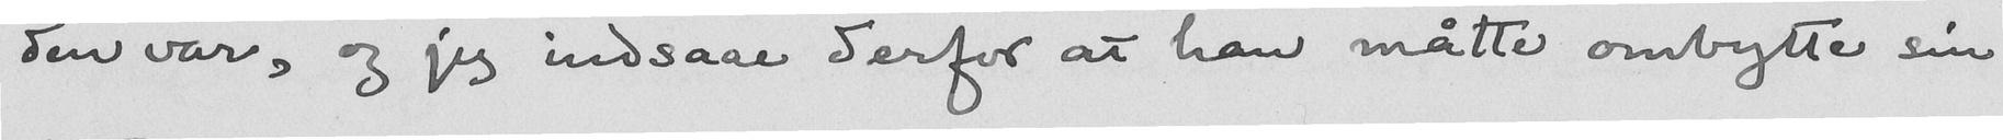den var, og jeg indsaae derfor at han måtte ombytte sin
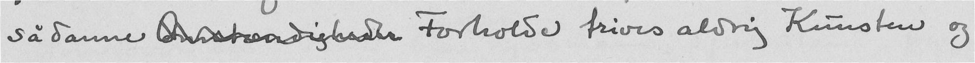sådanne Anstændigheder Forholde trives aldrig Kunsten og
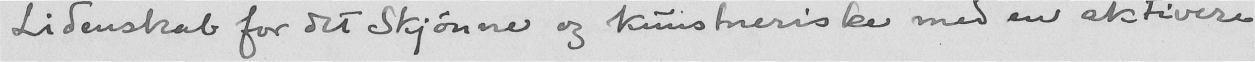Lidenskab for det skjønne og kunstneriske med en aktivere
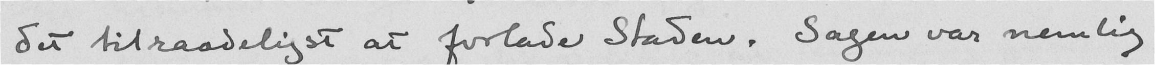det tilraadeligst at forlade Staden. Sagen var nemlig
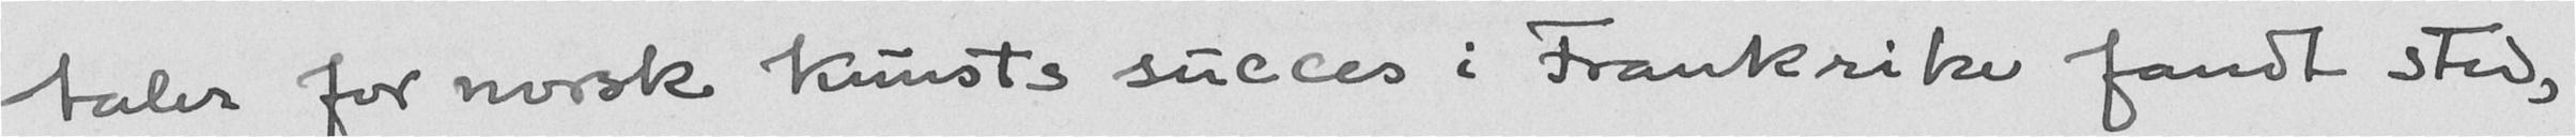taler for norsk kunsts succes i Frankrike fandt sted,
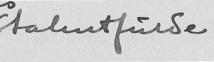talentfulde
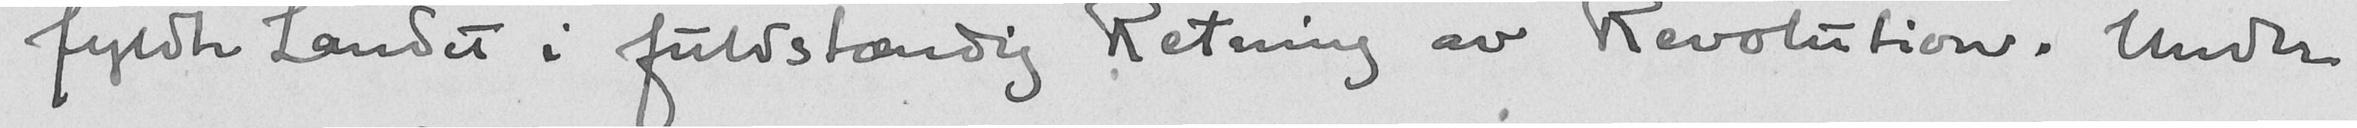fyldte landet i fuldstændig Retning av Revolution. Under
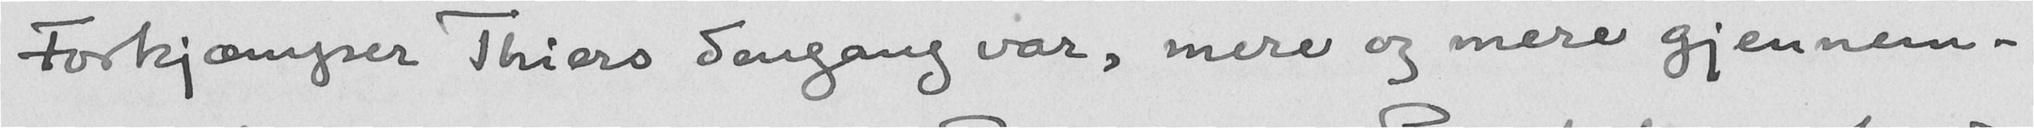Forkjæmper Thiers dengang var, mere og mere gjennem-
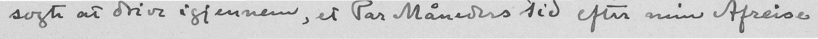sogte at drive igjennem, et Par Måneders tid efter min Afreise
